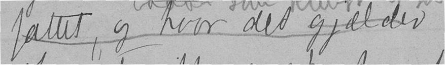fattet, og hvor det gjælder
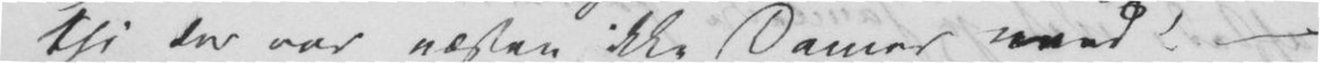thi der var næsten ikke Damer med! –
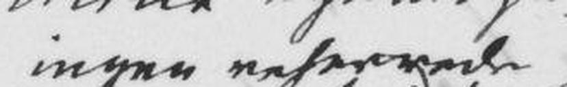ingen reserve[re]de
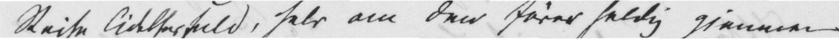Reise lidelsesfuld, selv om den fører heldig gjennem
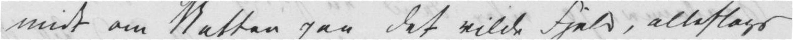midt om Natten paa det vilde Fjeld, alleslags
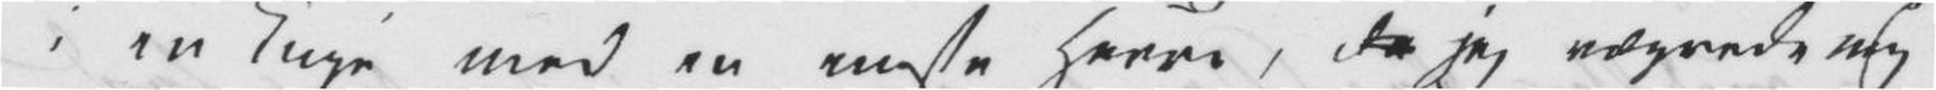i en Kupé med en eneste Herre, da jeg vægrede mig
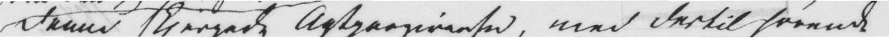Denne skjerpede Agtpaagivenhed, med dertil hørende
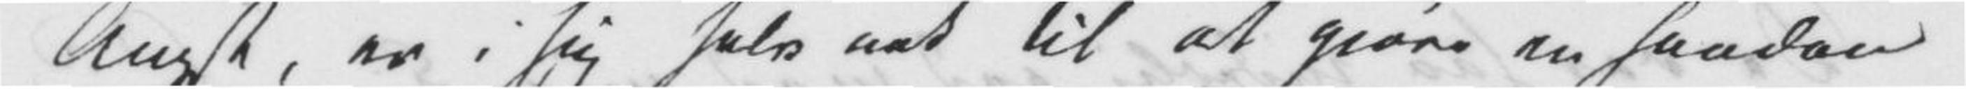Angst, er i sig selv nok til at gjøre en saadan
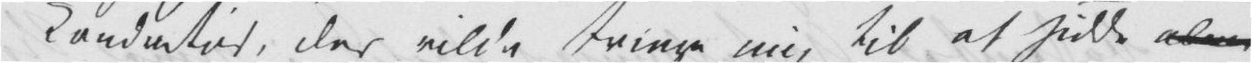Konductør, der vilde tvinge mig til at sidde alene
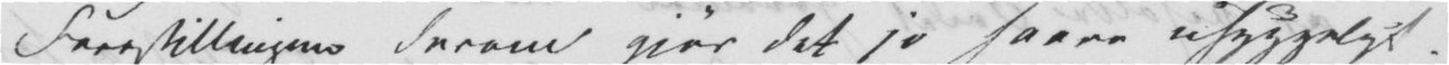Forestillingen derom gjør det jo saare uhyggeligt.
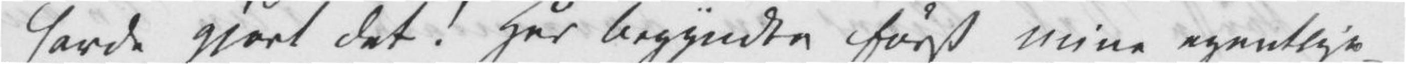havde gjort det! Her begyndte først mine egentlige
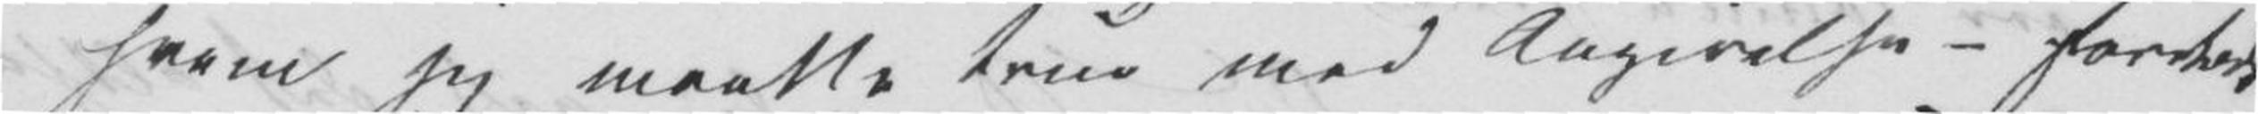hvem jeg maatte true med Angivelse – foretrak
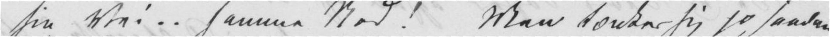sin Vei! .. Samme Nød! Man tænker sig jo saadan
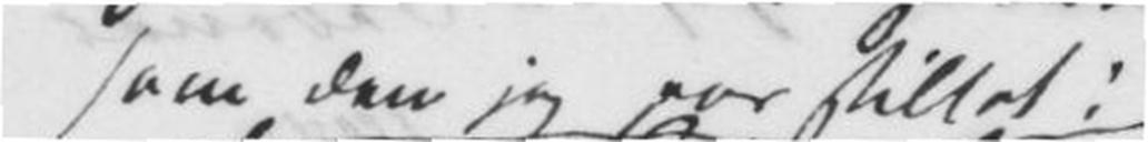som den jeg var stillet i[.]
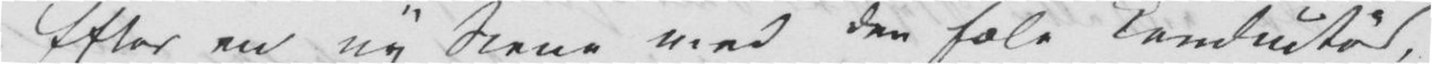Efter en ny Scene med den fæle Konductør,
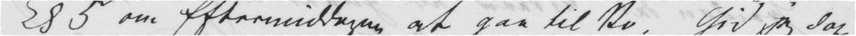Kl 5 om Eftermiddagen at gaa til Ro. Gid jeg dog
